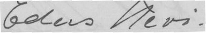Eders Nevø
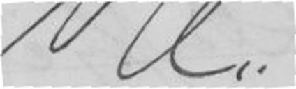Ole.
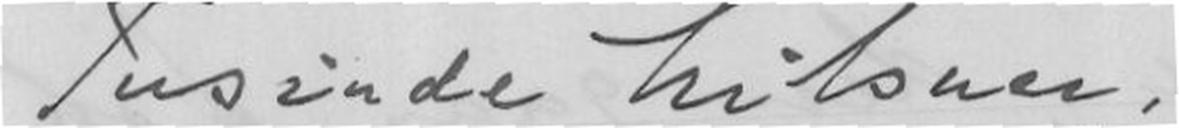Tusinde hilsener
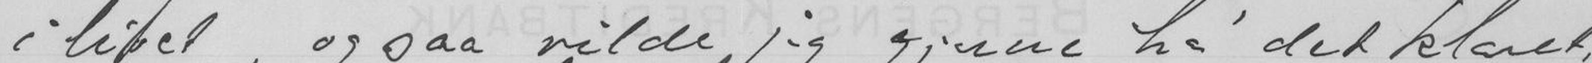i livet, og saa vilde jeg gjerne ha' det klaret.
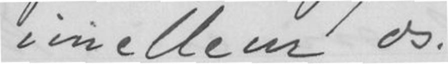imellem os.
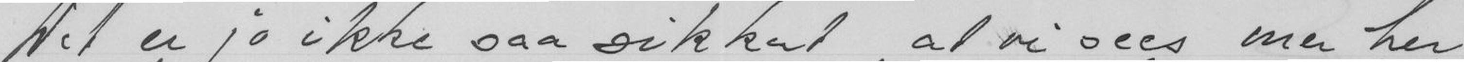Det er jo ikke saa sikkert, at vi sees mer her
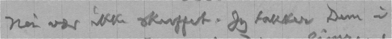Nei vær ikke skuffet. Jeg takker Dem i
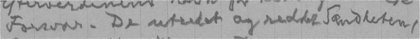Forsvar. De utredet og reddet Sandheten,
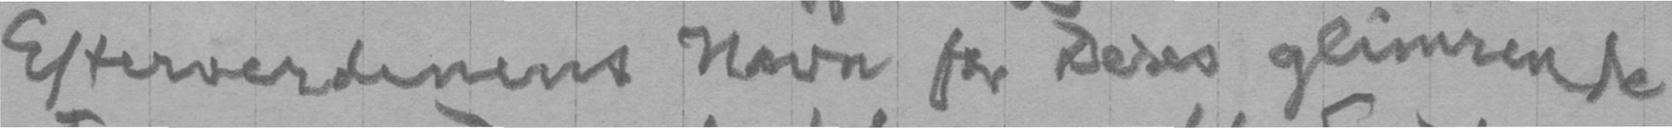Efterverdenens Navn for Deres glimrende
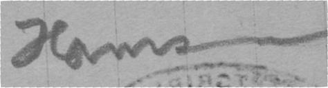Hamsun
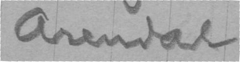Arendal
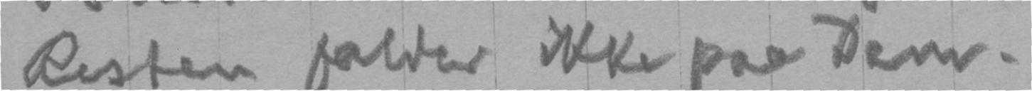Resten falder ikke paa Dem.
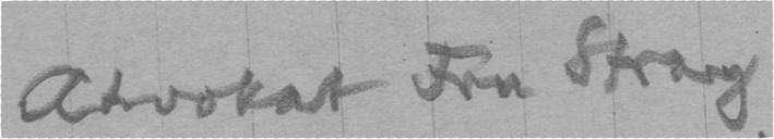Advokat Fru Stray
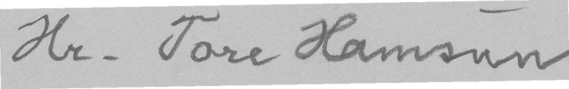Hr. Tore Hamsun
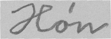Høn
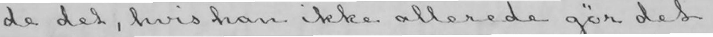de det, hvis han ikke allerede gør det.
